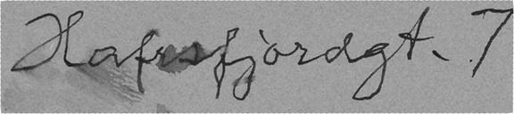Hafrsfjordgt. 7
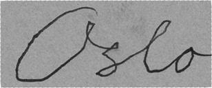Oslo
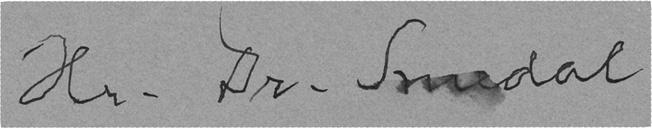Hr. Dr. Smedal
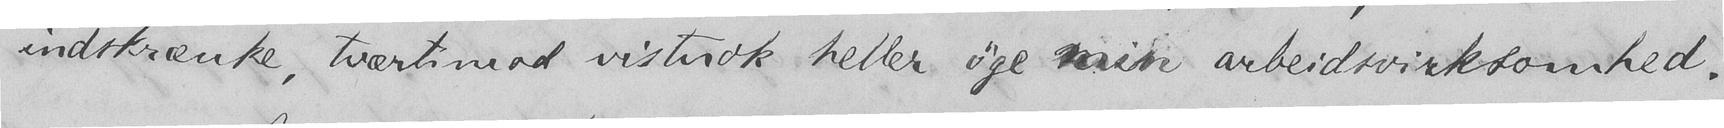indskrænke, tværtimod vistnok heller øge min arbeidsvirksomhed.
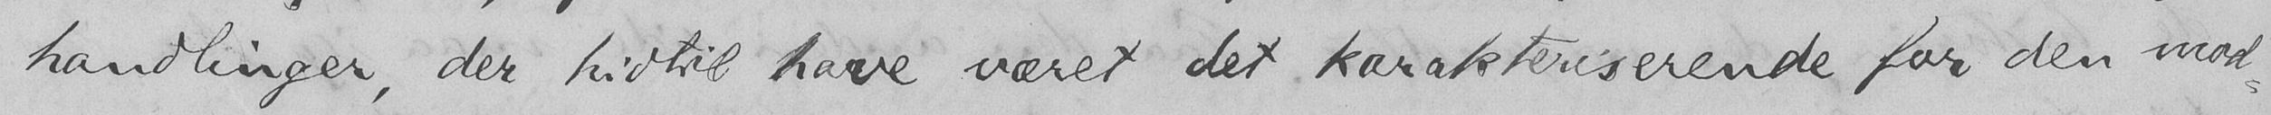handlinger, der hidtil have været det karakteriserende for den mod-
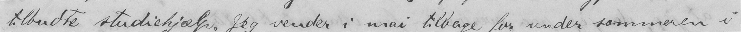tilbudte studiehjælp. Jeg vender i mai tilbage for under sommeren i
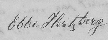Ebbe Hertzberg.
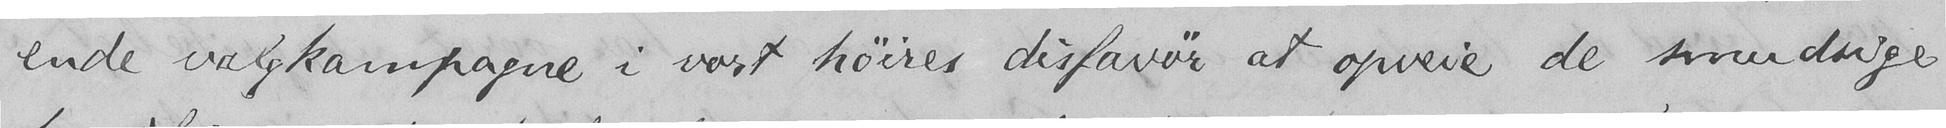ende valgkampagne i vort høires disfavør at opveie de smudsige
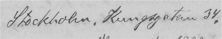Stockholm, Kungsgatan 34,
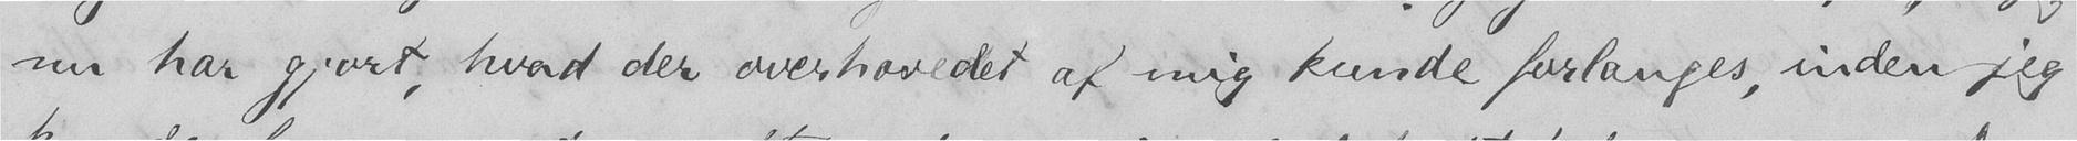nu har gjort, hvad der overhovedet af mig kunde forlanges, inden jeg
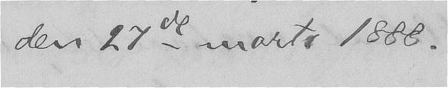den 27de marts 1888.
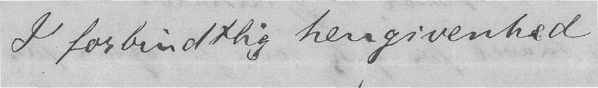I forbindtlig hengivenhed
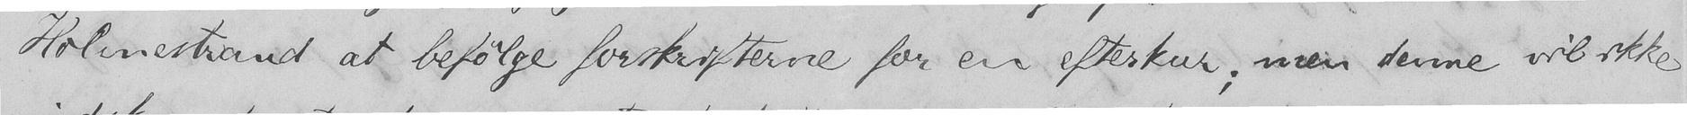Holmestrand at befølge forskrifterne for en efterkur; men denne vil ikke
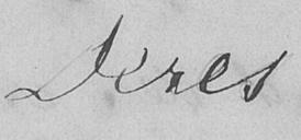Deres
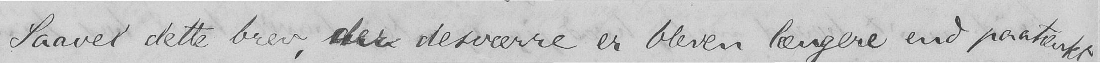Saavel dette brev, der desværre er bleven længere end paatænkt,
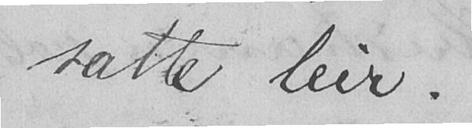satte leir.
